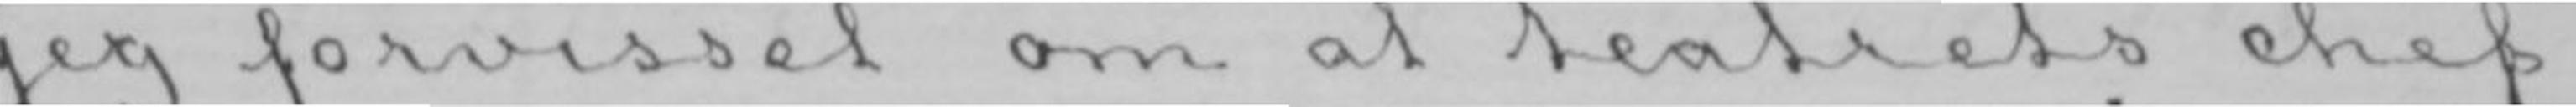jeg forvisset om at teatrets chef
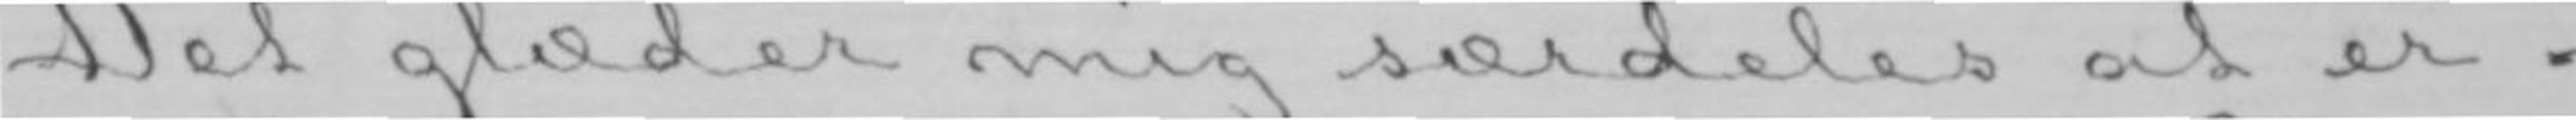Det glæder mig særdeles at er-
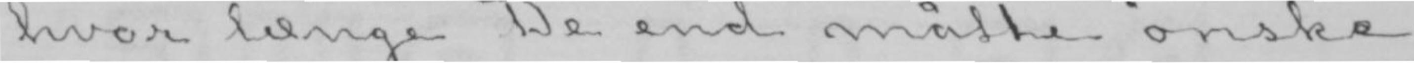hvor længe De end måtte ønske
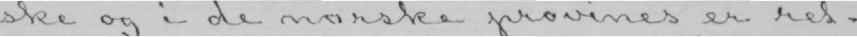ske og i de norske provines er ret-
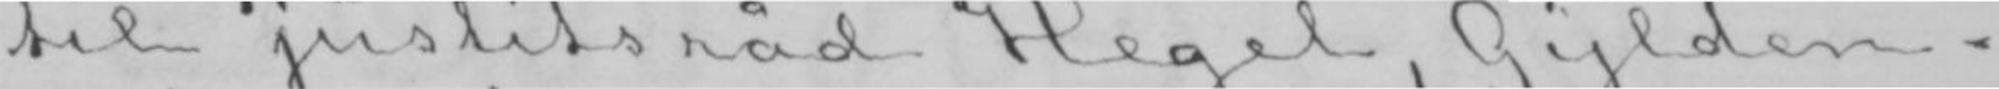til justitsråd Hegel, Gylden-
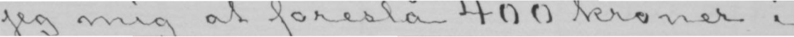jeg mig at foreslå 400 kroner i
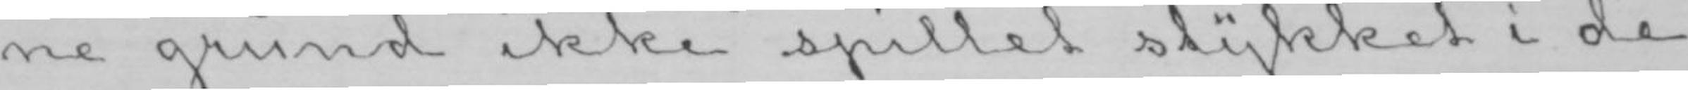ne grund ikke spillet stykket i de
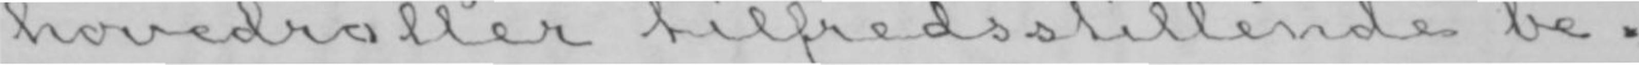hovedroller tilfredsstillende be-
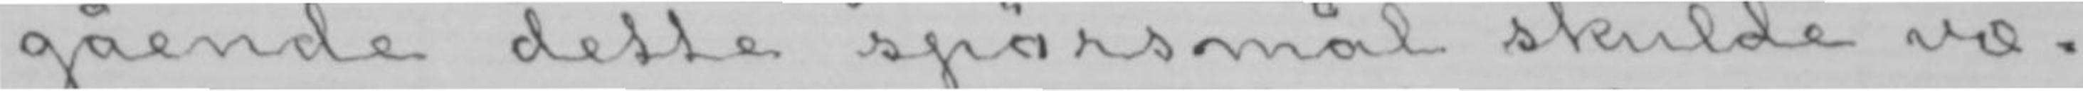gående dette spørsmål skulde væ-
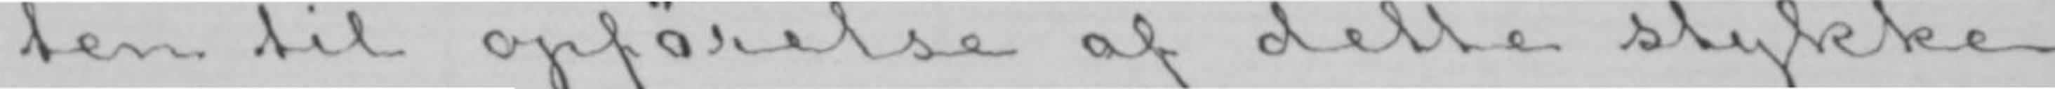ten til opførelse af dette stykke
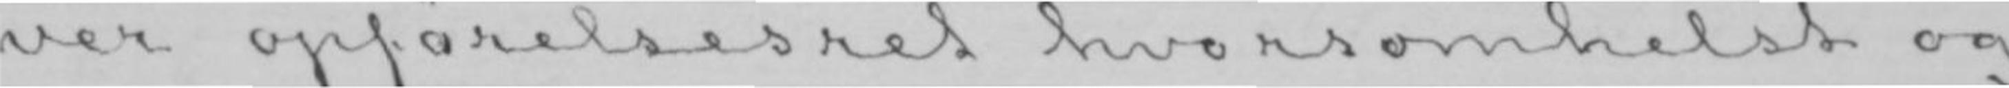ver opførelsesret hvorsomhelst og
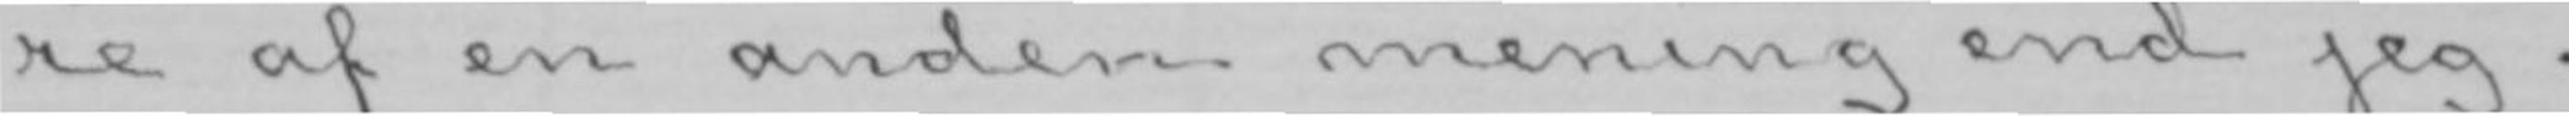re af en anden mening end jeg.
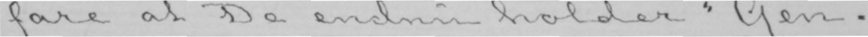fare at De endnu holder «Gen-
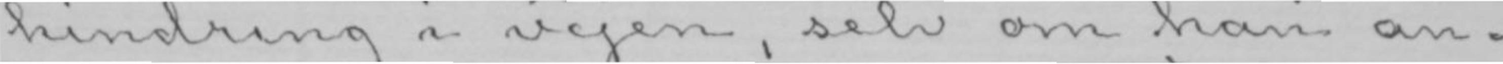hindring i vejen, selv om han an-
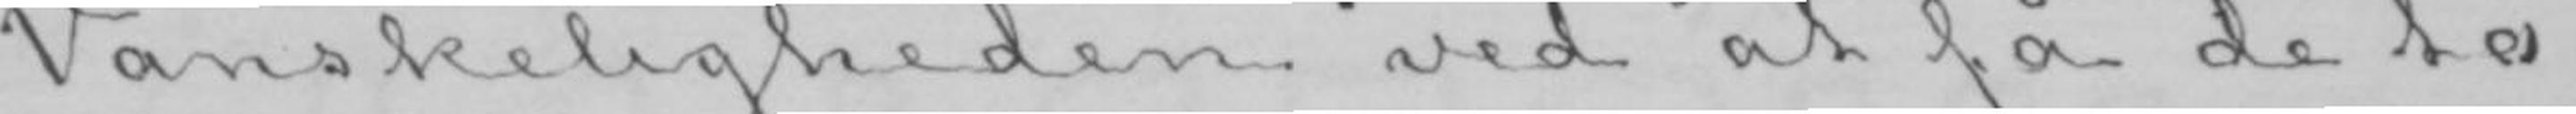Vanskeligheden ved at få de to
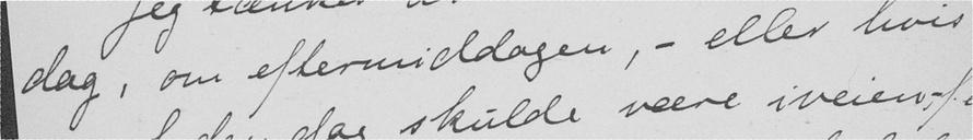dag, om eftermiddagen, - eller hvis
